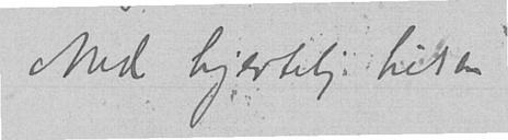Med hjertelig hilsen
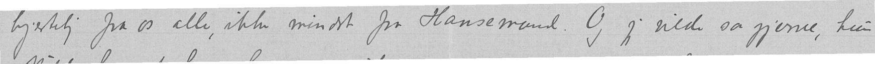hjertelig fra os alle, ikke mindst fra Hansemand. Og jeg vilde saa gjerne, hun
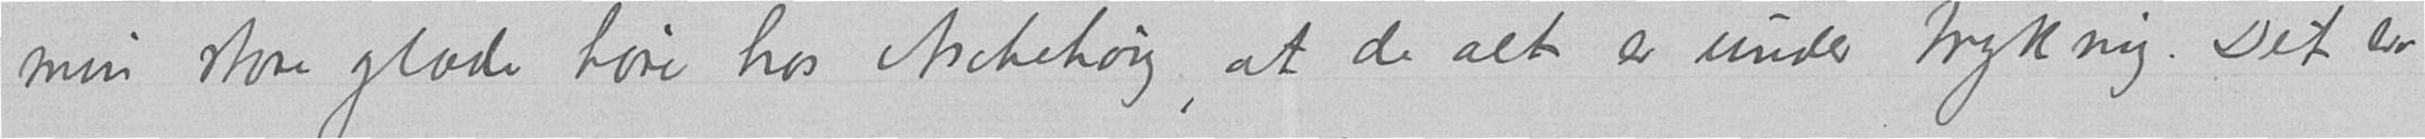min store glæde høre hos Aschehoug, at de alt er under trykning. Det er
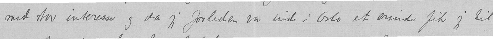med stor interesse og da jeg forleden var inde i Oslo et erinde fik jeg til
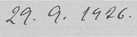29.9.1926.
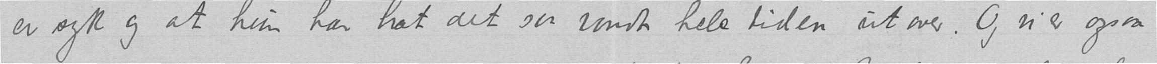er syk og at hun har hat det saa vondt hele tiden utover. Og vi er ogsaa
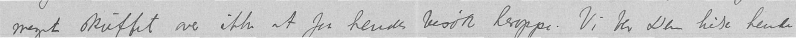meget skuffet over ikke at faa hendes besøk heroppe. Vi ber Dem hilse hende
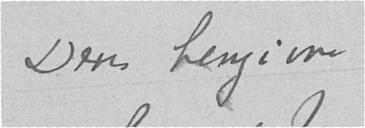Deres hengivne
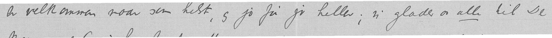er velkommen naar som helst, og jo før jo heller; vi glæder os alle til De
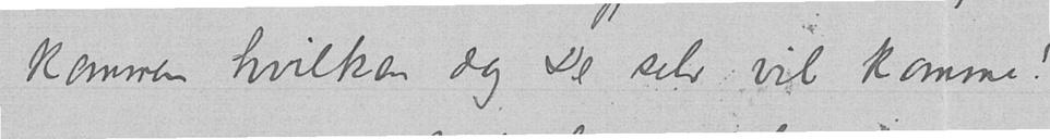kommen hvilken dag De selv vil komme!
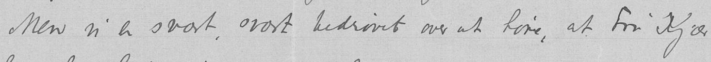Men vi er svært, svært bedrøvet over at høre, at Fru Kjær
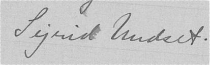Sigrid Undset.
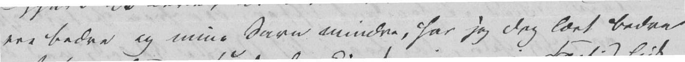ere bedre og mine Savn mindre, har jeg dog lært bedre
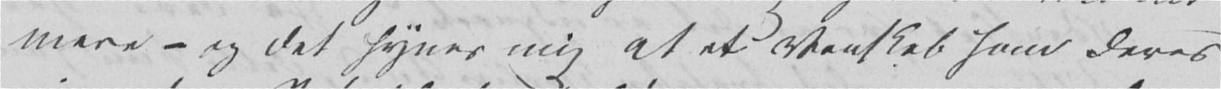mere – og det synes mig at et Venskab som Deres
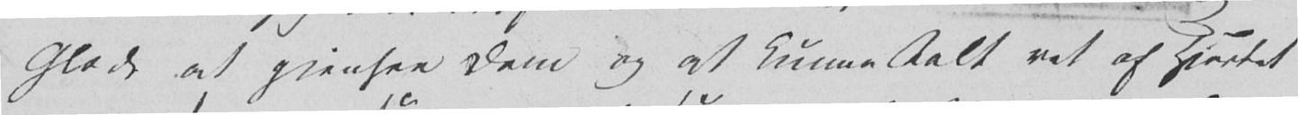Glæde at gjensee Dem og at kunne talt ret af Hjertet
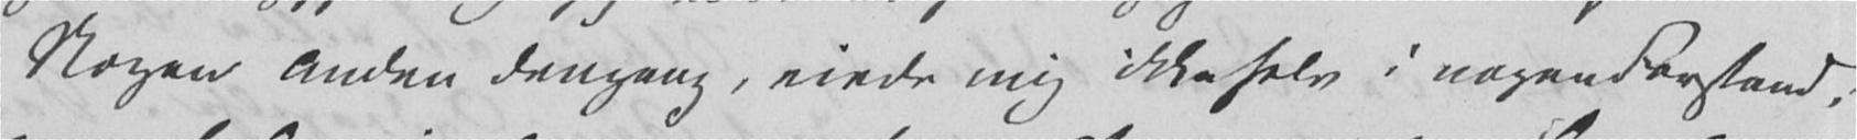Nogen Anden dengang, eiede mig ikke selv i nogen Forstand,
